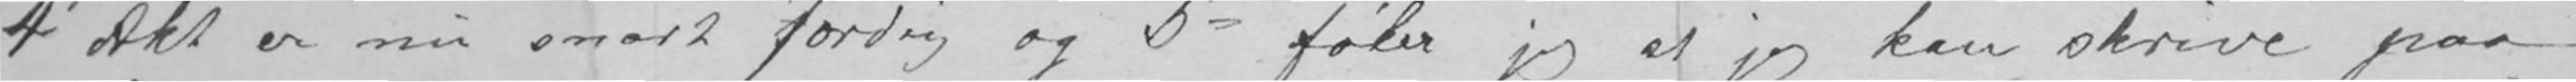4de Akt er nu snart færdigFærdig og 5te føler jeg at jeg kan skrive paa
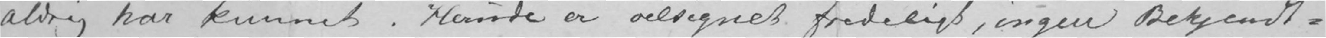aldrig har kunnet. Herude er velsignet fredeligt, ingen Bekjendt-
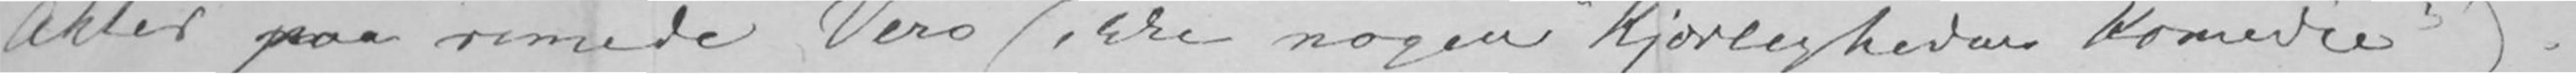Akter paa rimede Vers (ikke nogen «Kjærlighedens Komedie»).
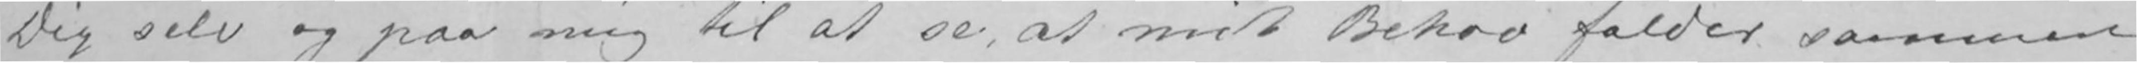Dig selv og paa mig til at se, at mit Behov falder sammen
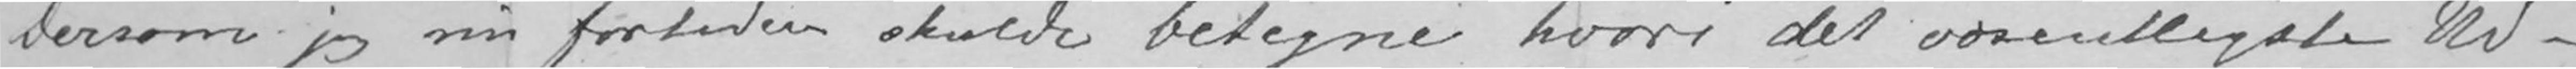- Dersom jeg nu fortiden skulde betegne hvori det væsentligste Ud-
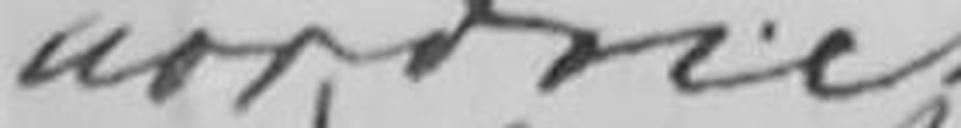uordnet
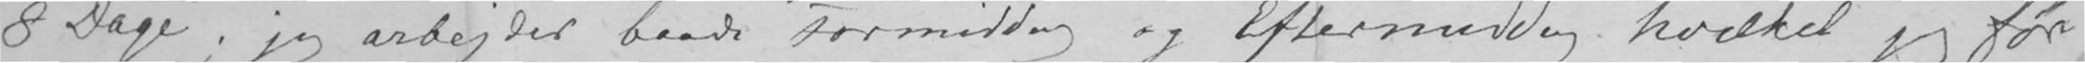8 Dage; jeg arbejder baade Formiddag og Eftermiddag hvilket jeg før
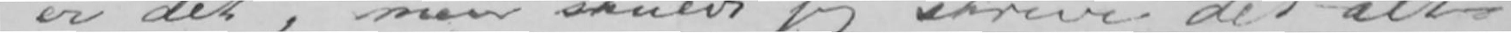er det, men skulde jeg skrive det alt-
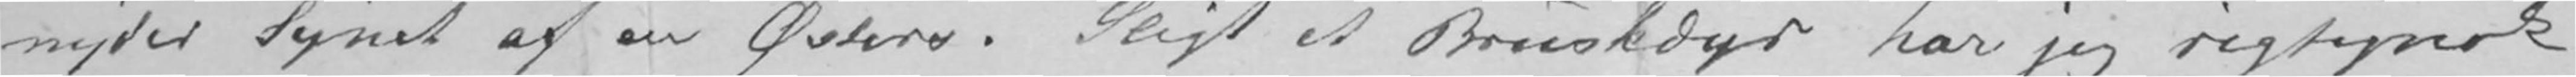nyder Synet af en Østers. Sligt et Bruskdyr har jeg rigtignok
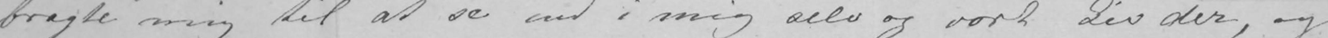bragte mig til at se ind i mig selv og vort Liv der, og
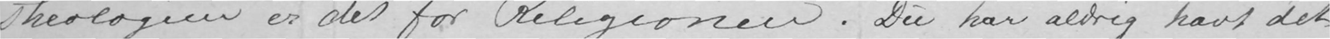Theologien er det for Religionen. Du har aldrig havt det
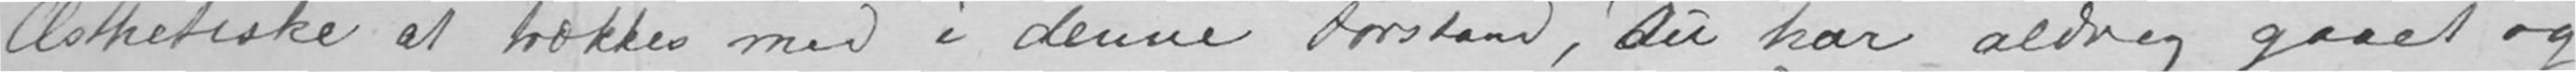Æsthetiske at trækkes med i denne Forstand, Dudu har aldrig gaaet og
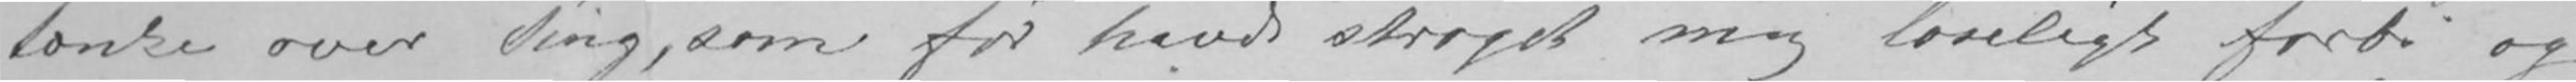tænke over Ting, som før havde strøget mig løseligt forbi og
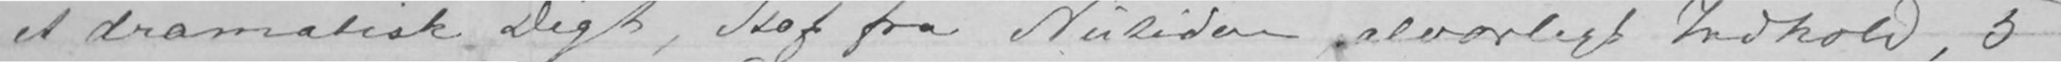et dramatisk Digt, Stof fra Nutiden, alvorligt Indhold, 5
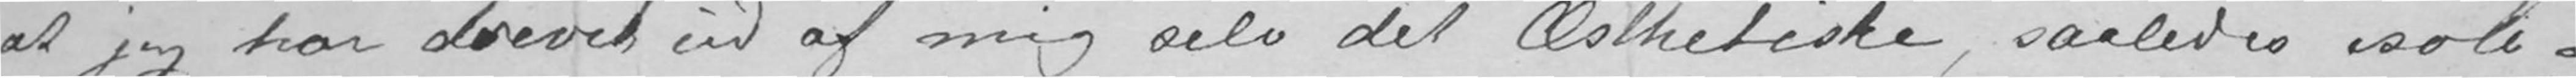at jeg har drevet ud af mig selv det Æsthetiske, saaledes isole-
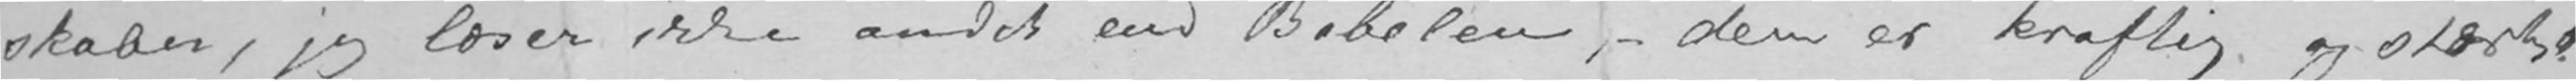skaber, jeg læser ikke andet end Bibelen,- den er kraftig og stærk!
